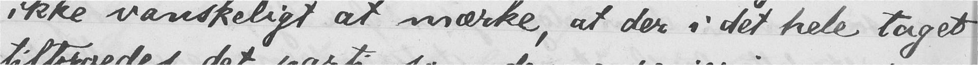ikke vanskeligt at mærke, at der i det hele taget
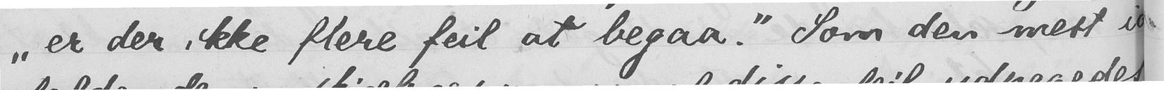"er der ikke flere feil at begaa." Som den mest iøine-
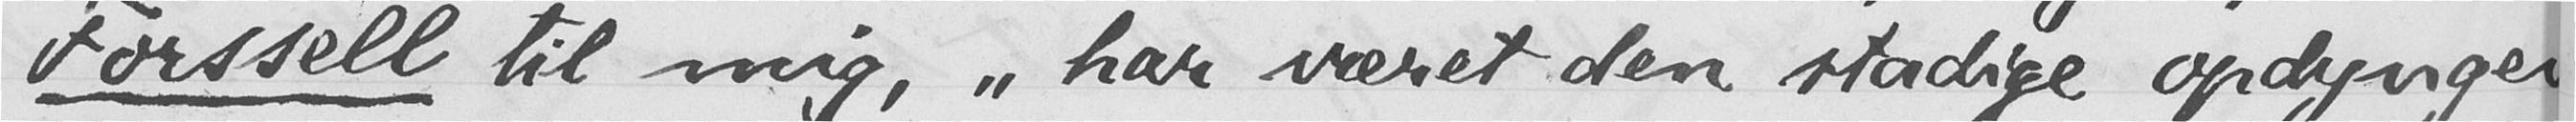Forssell til mig, "har været den stadige opdyngen
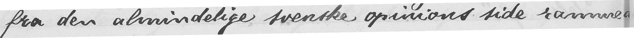fra den almindelige svenske opinions side rammede
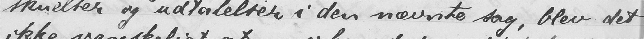skuelser og udtalelser i den nævnte sag, blev det
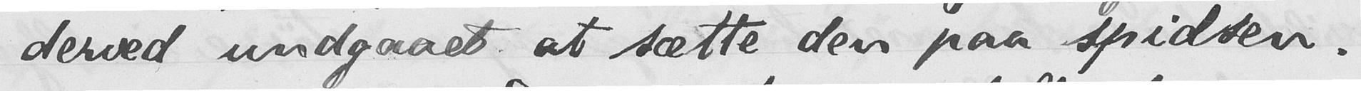derved undgaaet at sætte den paa spidsen.
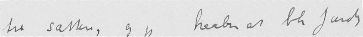tre sættere, og jeg haaber at bli færdig
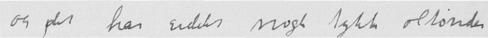og det har siddet nogle tykke øltønder
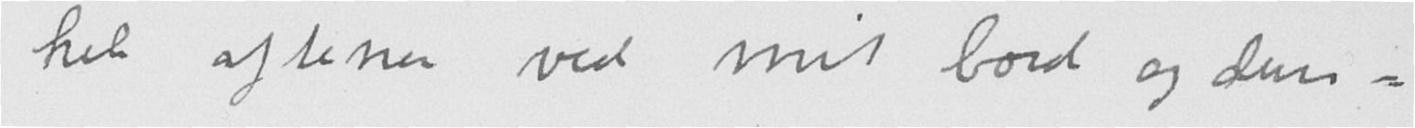hele aftenen ved mit bord og dun-
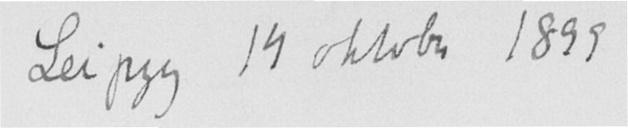Leipzig 19 oktober 1899
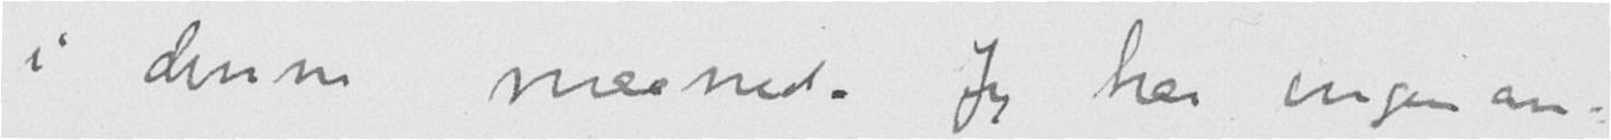i denne maaned. Jeg har ingen an-
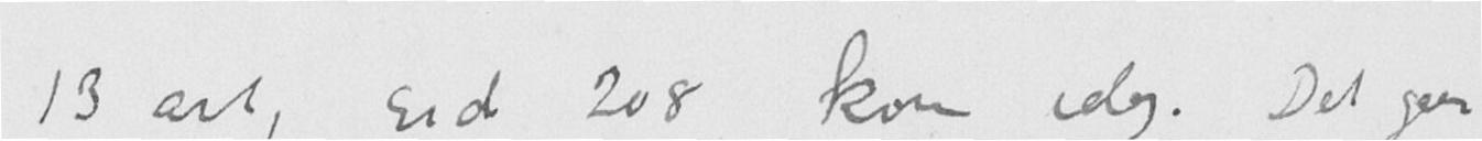13 ark, side 208 kom idag. Det gaar
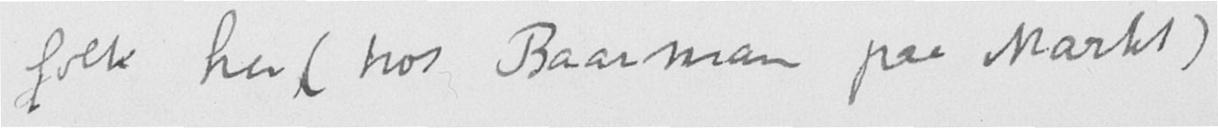folk her (hos Baarman paa Markt)
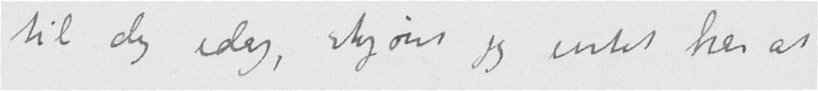til dig idag, skjønt jeg intet har at
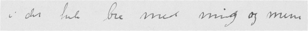i det hele bra med mig og mine
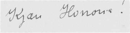Kjære Honoria!
